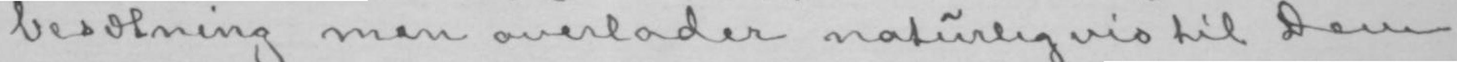besætning men overlader naturligvis til Dem
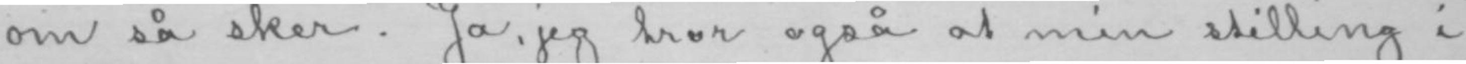om så sker. Ja, jeg tror også at min stilling i
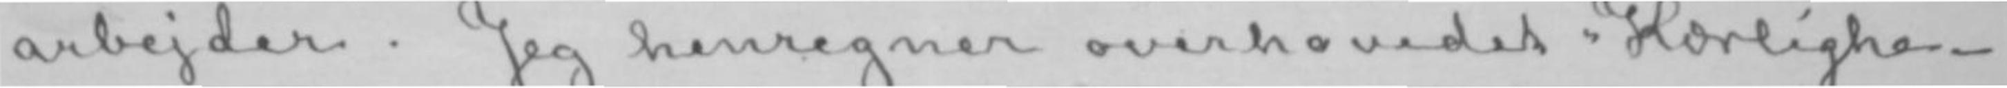arbejder. Jeg henregner overhovedet «Kærlighe-
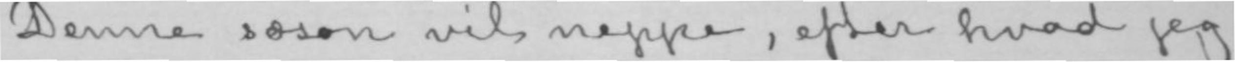Denne sæson vil neppe, efter hvad jeg
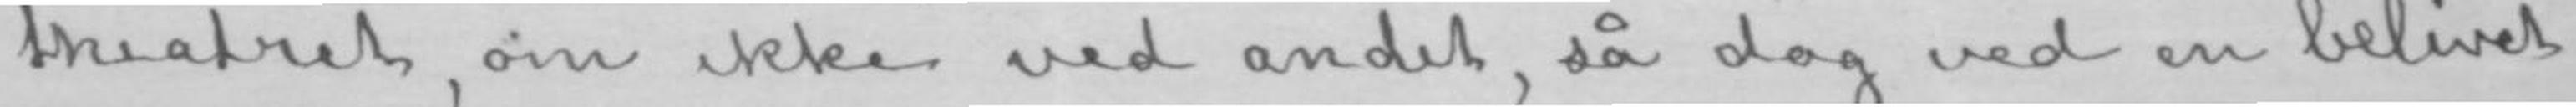theatret, om ikke ved andet, så dog ved en belivet
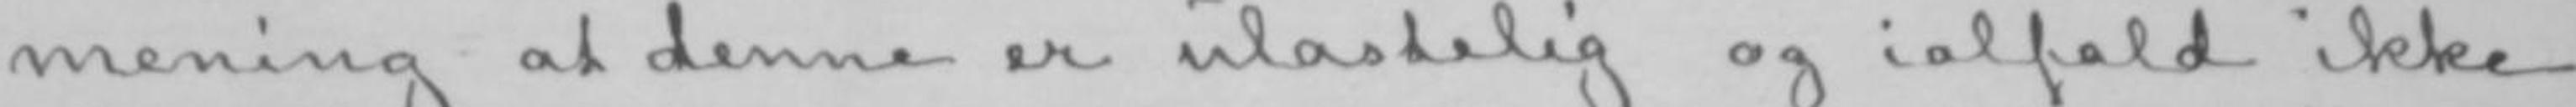mening at denne er ulastelig og ialfald ikke
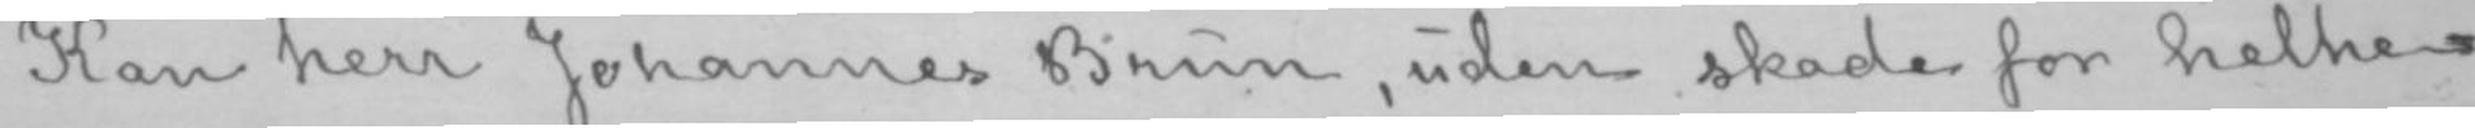Kan herr Johannes Brun, uden skade for helhe-
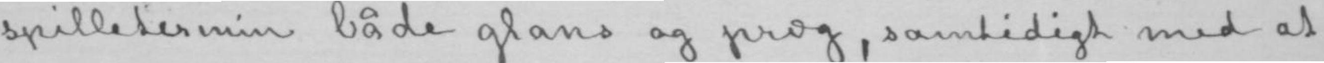spilletermin både glans og præg, samtidigt med at
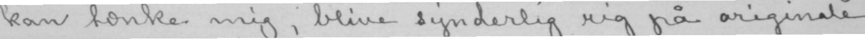kan tænke mig, blive synderlig rig på originale
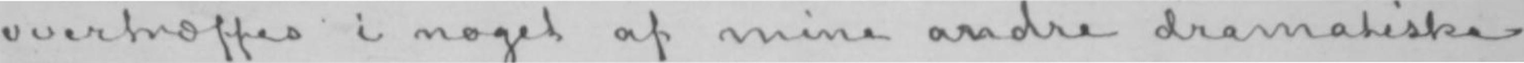overtræffes i noget af mine andre dramatiske
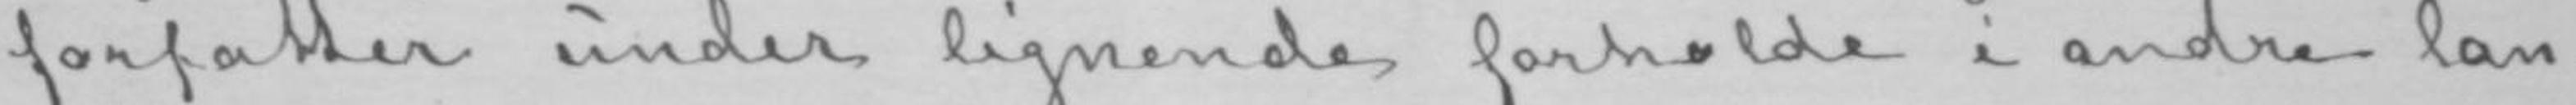forfatter under lignende forholde i andre lan-
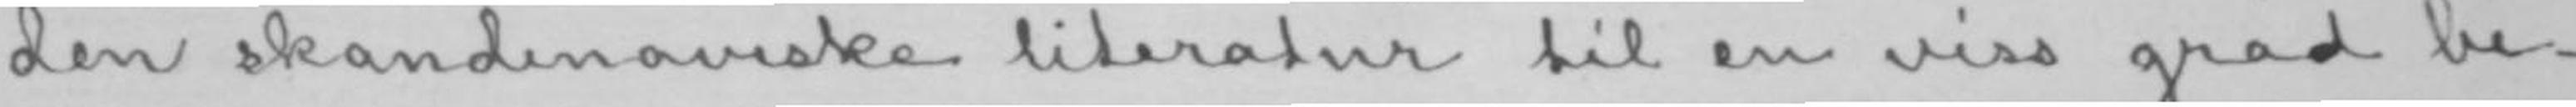den skandinaviske literatur til en viss grad be-
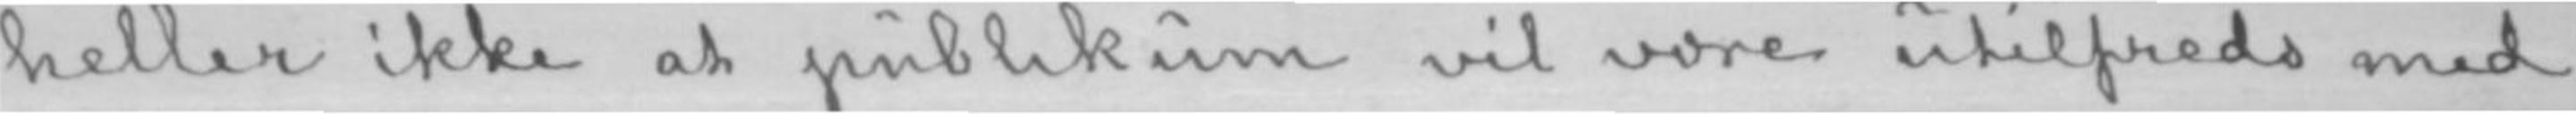heller ikke at publikum vil være utilfreds med
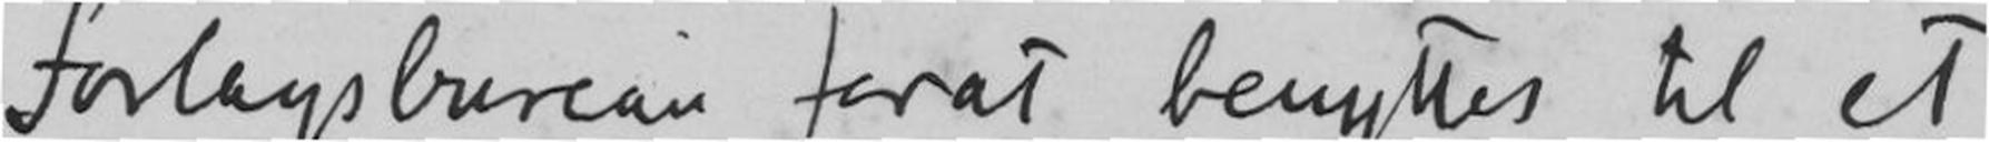forlagsbureau forat benyttes til et
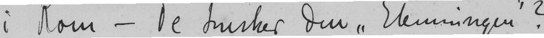i Roma- De Husker Den Skumingen?
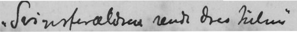Svigerforældrene sende deres hilsen
